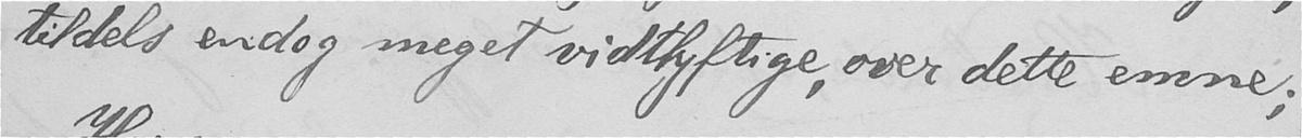tildels endog meget vidtlyftige, over dette emne;
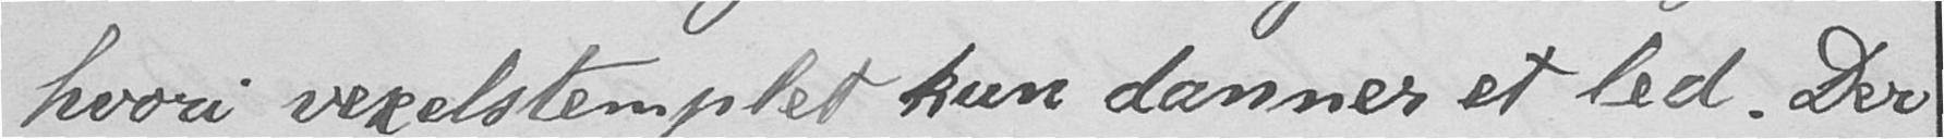hvori vexelstemplet kun danner et led. Der
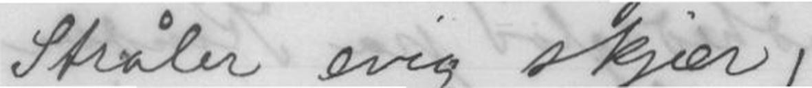Stråler evig skjær,
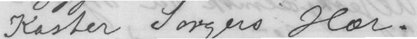Kaster Sorgers Hær
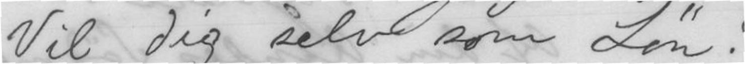Vil dig selv som Løn!
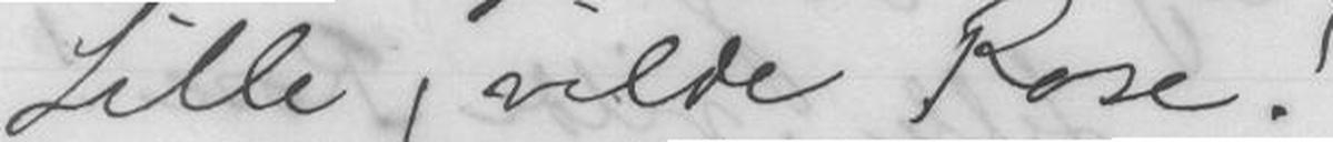Lille, vilde Rose!
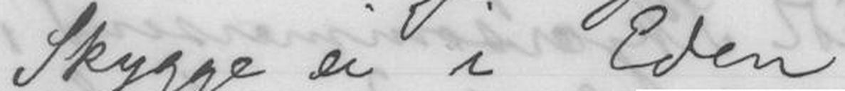Skygge ei i Eden
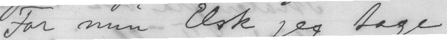For min Elsk jeg tage
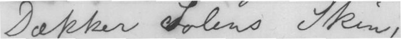Dækker Solens Skin,
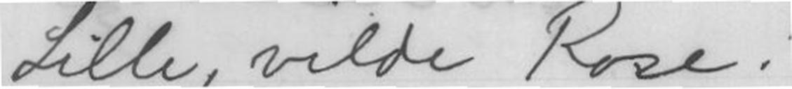Lille, vilde Rose!
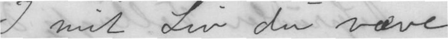I mit Liv du være
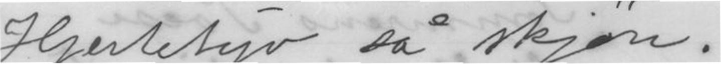Hjertetyv så skjøn.
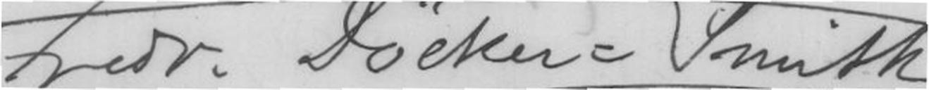Fredr. Döcker=Smith
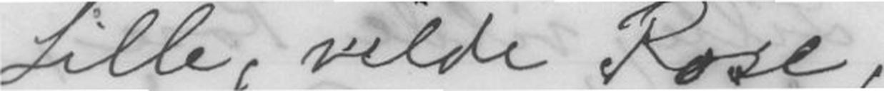Lille, vilde Rose,
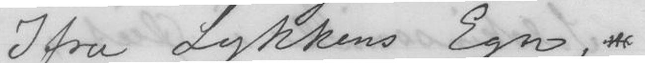Ifra Lykkens Egn,
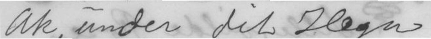Ak, under dit Hegn
